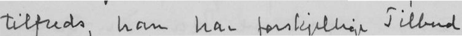tilfreds, han har forskjellige tilbud
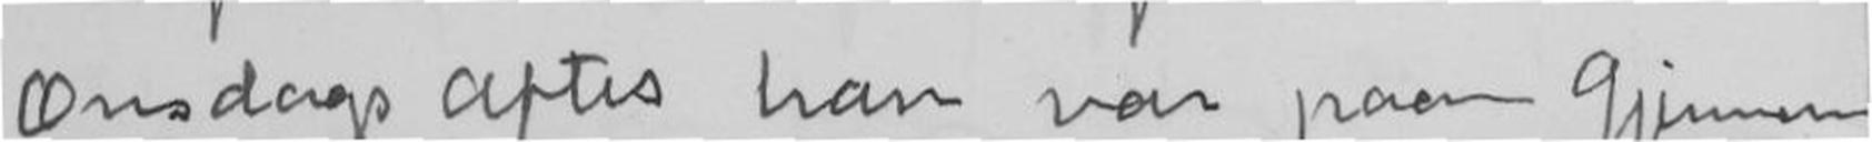Onsdags Aften han var paa gjennom-
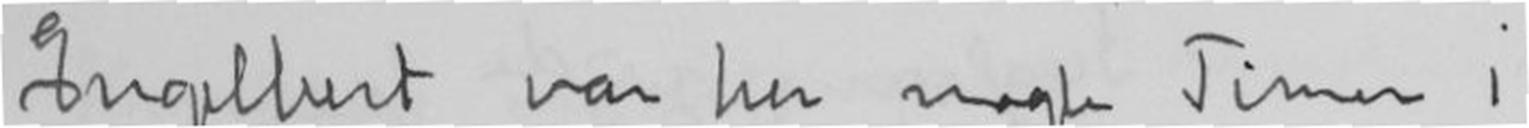Engelbert var her nogle Timer i
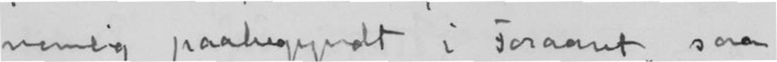nemlig paabegyndt i Foraaret, saa
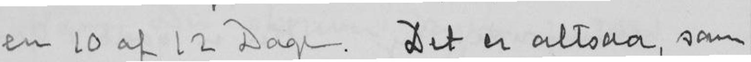en 10 af 12 Dage. Det er altsaa, som
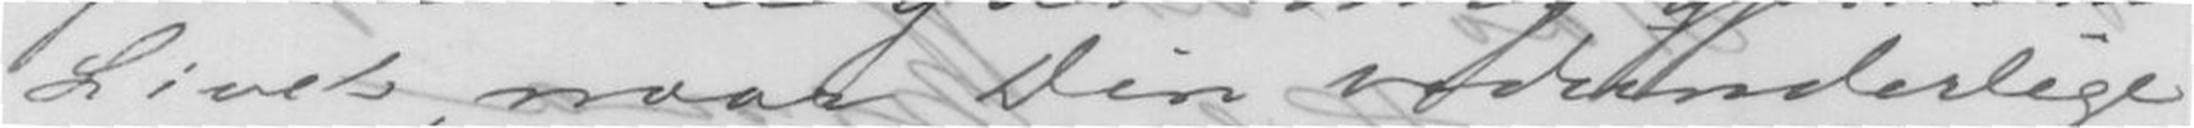Livet, naar Din vidunderlige
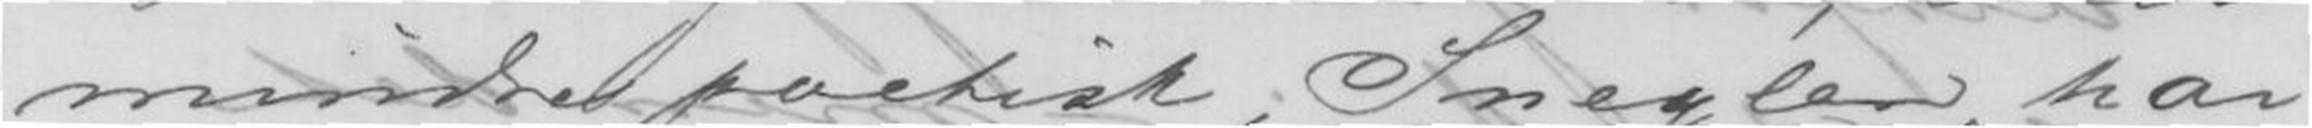mindre poetisk, Sneglen, har
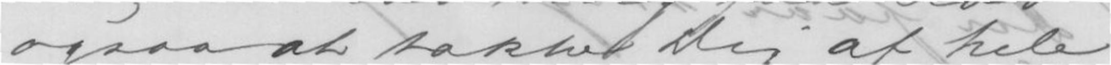ogsaa at takke Dig af hele
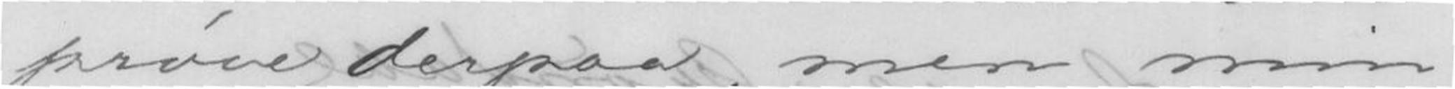prøve derpaa, men min
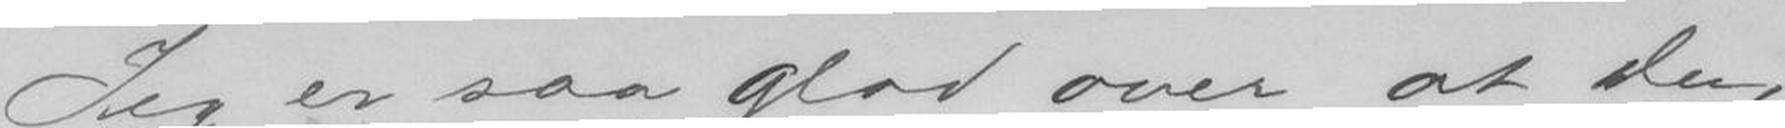Jeg er saa glad over at Du,
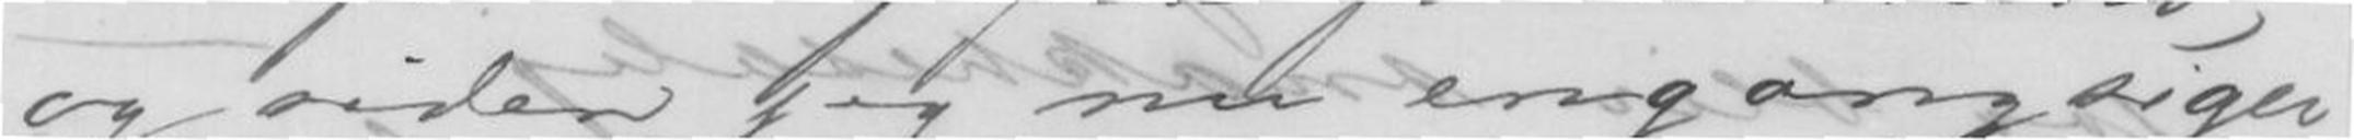og siden jeg nu engang siger
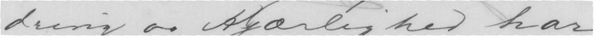dring og Kjærlighed har
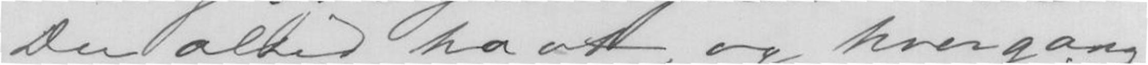Du altid havt, og hvergang
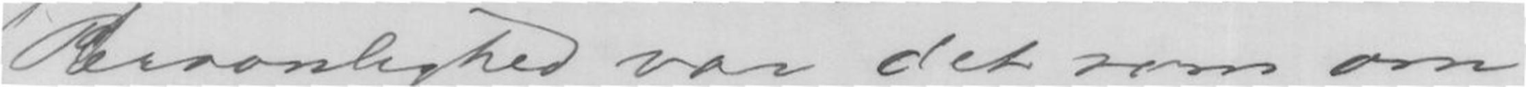Personlighed var det som om
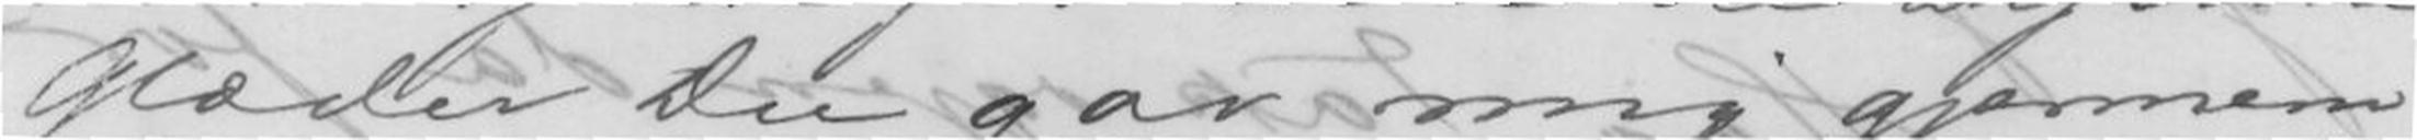Glæder Du gav mig gjennem
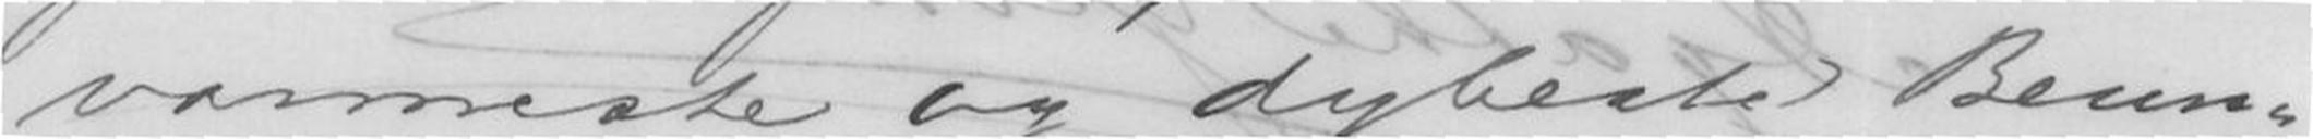varmeste og dybeste Beun-
